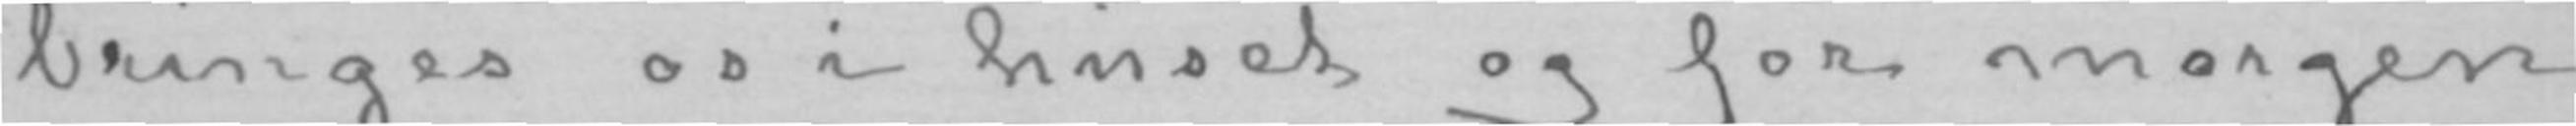bringes os i huset og for morgen
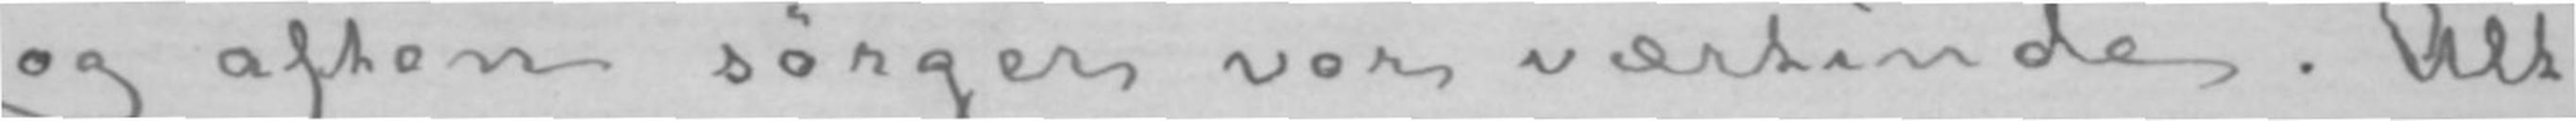og aften sørger vor værtinde. Alt
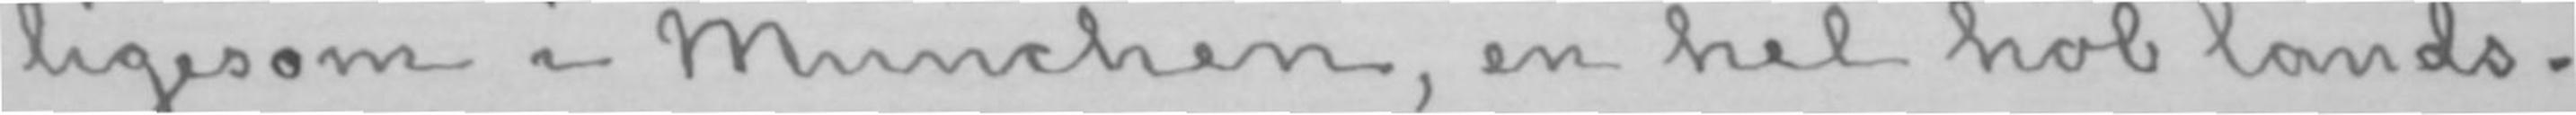ligesom i München, en hel hob lands-
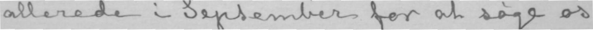allerede i September for at søge os
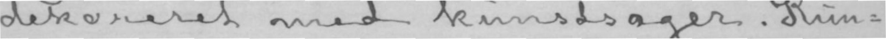dekoreret med kunstsager. Kun-
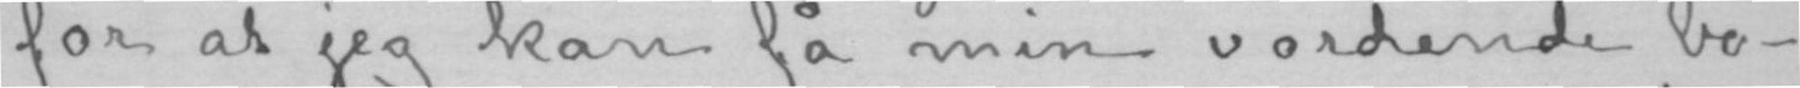for at jeg kan få min vordende bo-
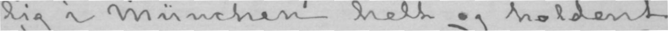lig i München helt og holdent
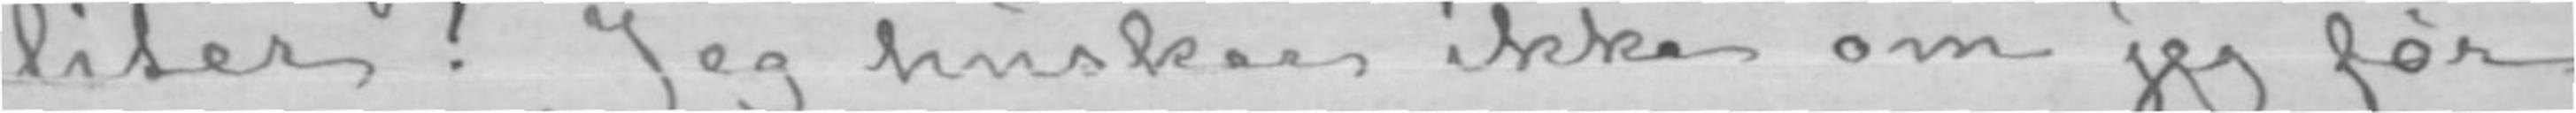liter! Jeg husker ikke om jeg før
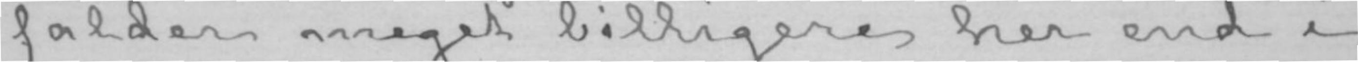falder meget billigere her end i
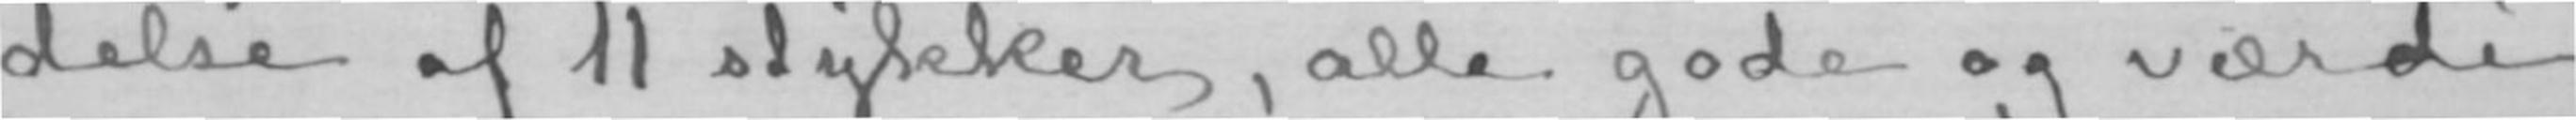delse af 11 stykker, alle gode og værdi-
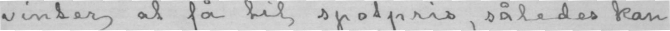vinter at få til spotpris, således kan
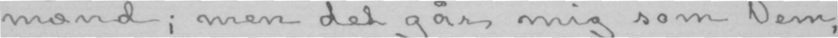mænd; men det går mig som Dem,
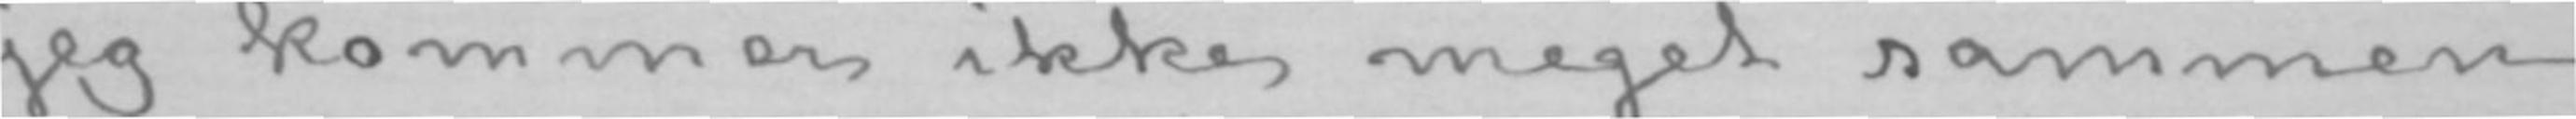jeg kommer ikke meget sammen
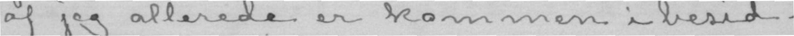af jeg allerede er kommen i besid-
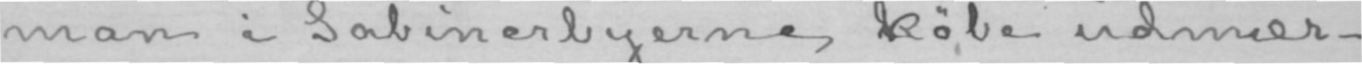man i Sabinerbyerne købe udmær-
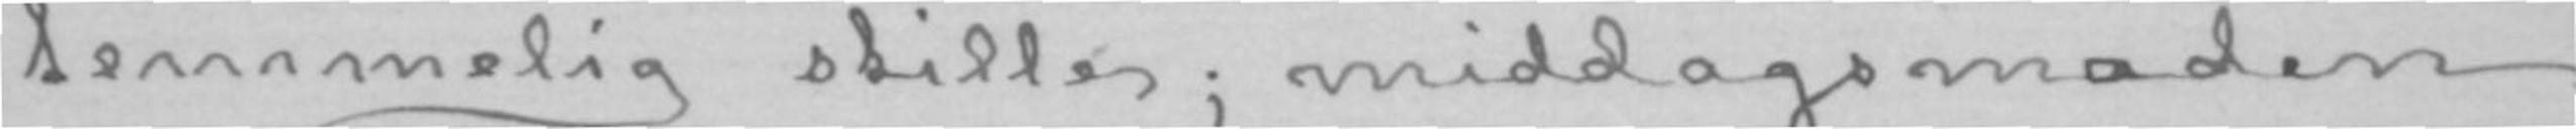temmelig stille; middagsmaden
